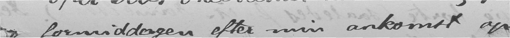g formiddagen efter min ankomst op
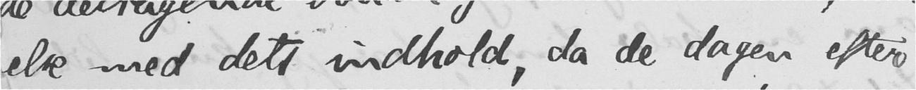else med dets indhold, da de dagen efter
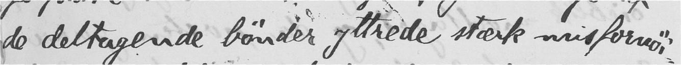de deltagende bønder yttrede stærk misfornøi-
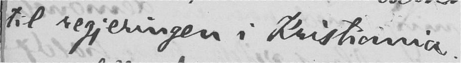til regjeringen i Kristiania.
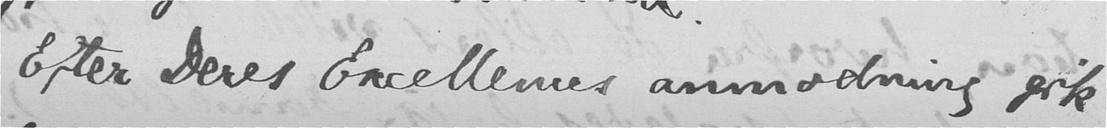Efter Deres Excellences anmodning gik
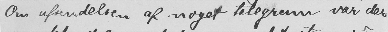Om afsendelsen af noget telegram var der
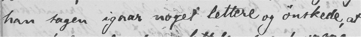han sagen igaar noget lettere og ønskede, at
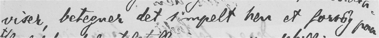viser, betegner det simpelt hen et forsøg paa
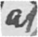at
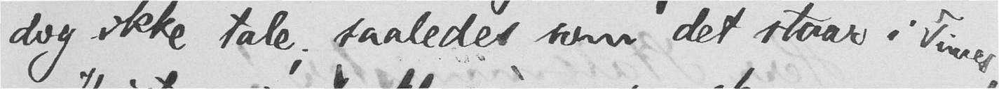dog ikke tale; saaledes som det staar i Times,
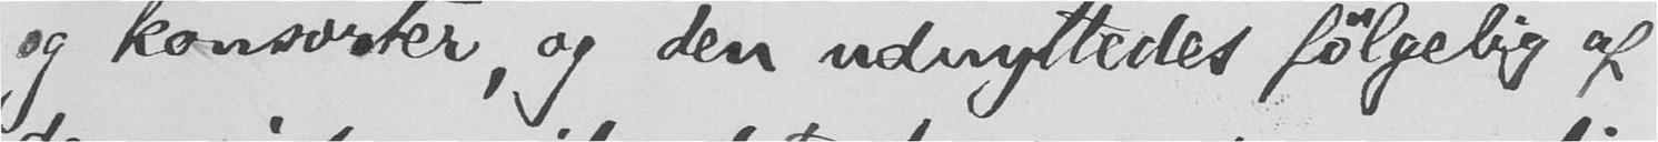og konsorter, og den udnyttedes følgelig af
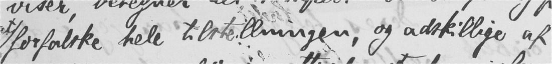forfalske hele tilstellningen, og adskillige af
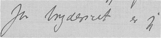for brydersiet er jeg
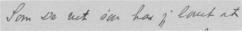Som De vet saa har jeg lovet at
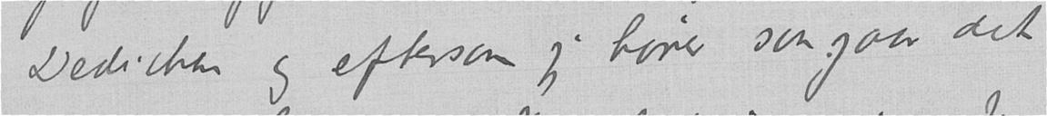Dedichen og eftersom jeg hører saa gaar det
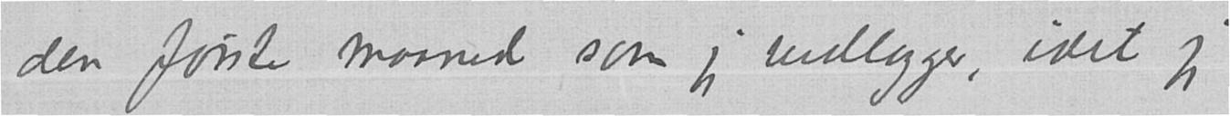den første maaned som jeg vedlegger, idet jeg
